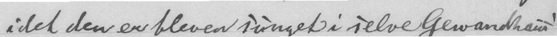idet den er bleven sunget i selve Gewandhaus
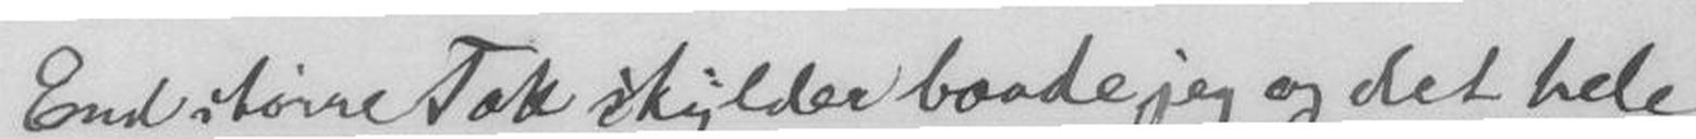End større Tak skylder baade jeg og det hele
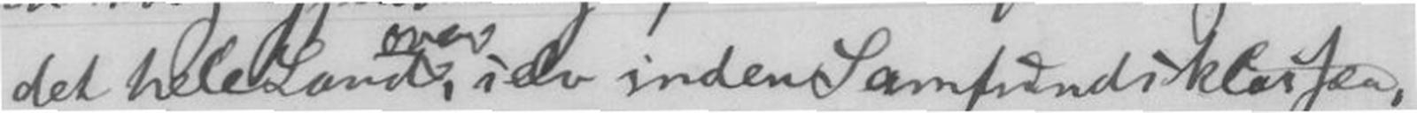det hele Land, over selv inden Samfundsklassen,
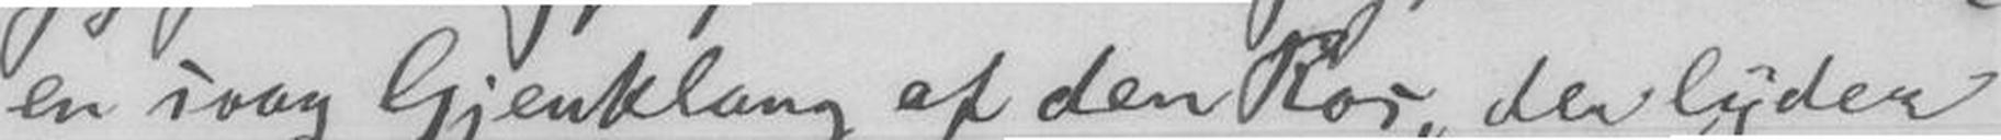en sang Gjenklang af den Ros, der lyder
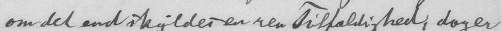om det end skylder en ren Tilfældighed, dog er
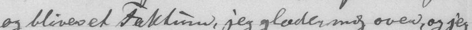og blives et Faktum, jeg glæder mig over, og jeg
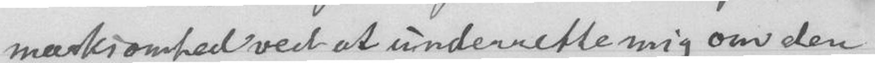mærksomhed ved at underrette mig om den
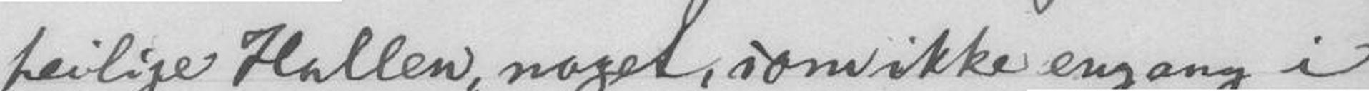heilige Hallen, noget, som ikke engang i
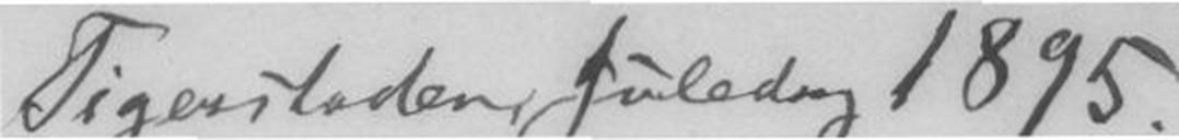Tigerstaden Juledag 1895.
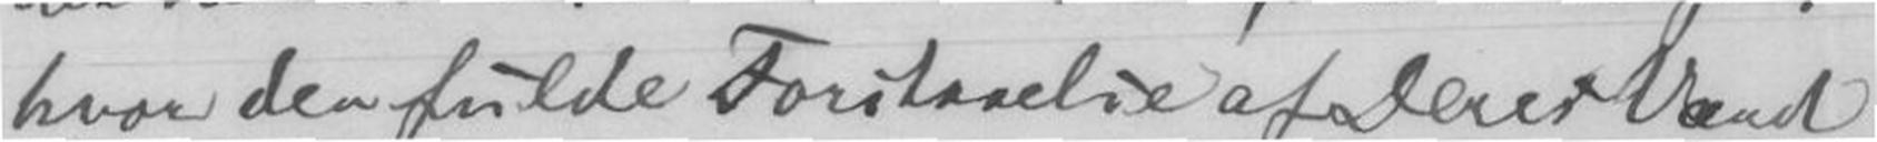hvor den fulde Forstaaelse af Deres Værd
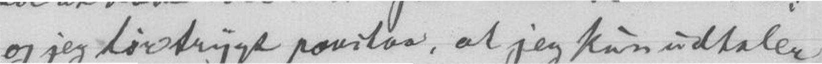og jeg tør trygt paastaa, at jeg kun udtaler
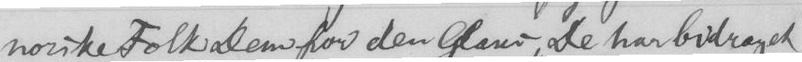norske Folk Dem for den Glans, De har bidraget
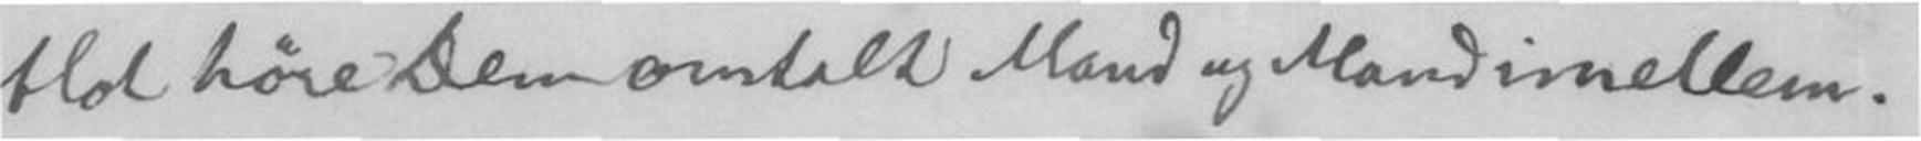blot høre Dem omtalt Mand og Mand imellem.
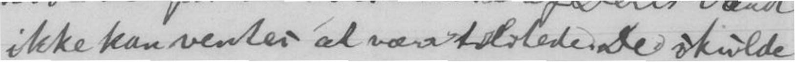ikke kan ventes at være tilstede. De skulde
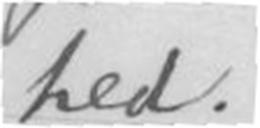hed.
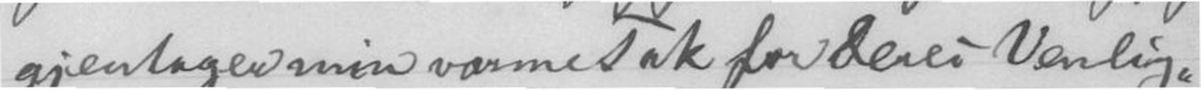gjentager min varme Tak for Deres Venlig-
